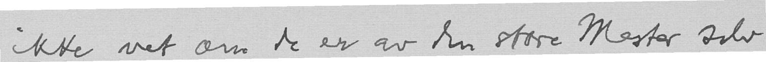ikke vet om de er av den store Mester selv
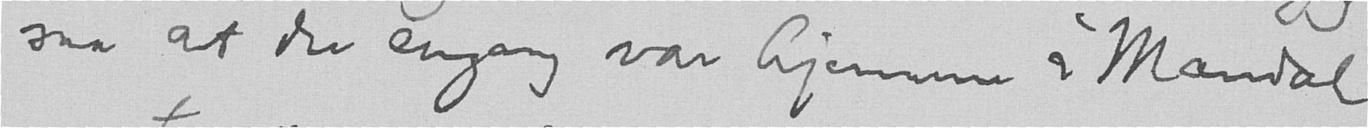saa at du engang var hjemme i Mandal
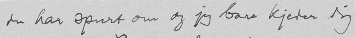du har spurt om og jeg bare kjeder dig
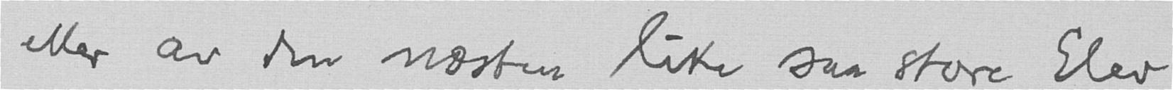eller av den næsten like saa store Elev
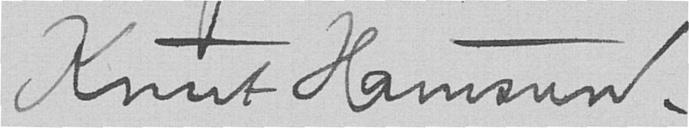Knut Hamsun.
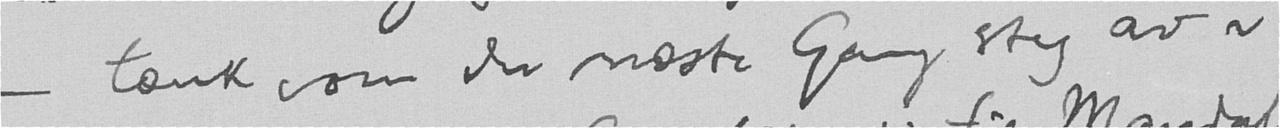- tænk om du næste Gang steg av i
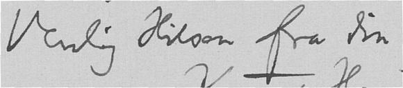Venlig Hilsen fra din
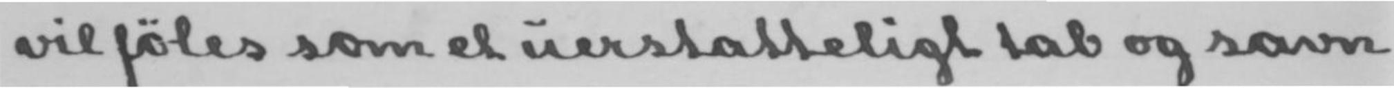vil føles som et uerstatteligt tab og savn
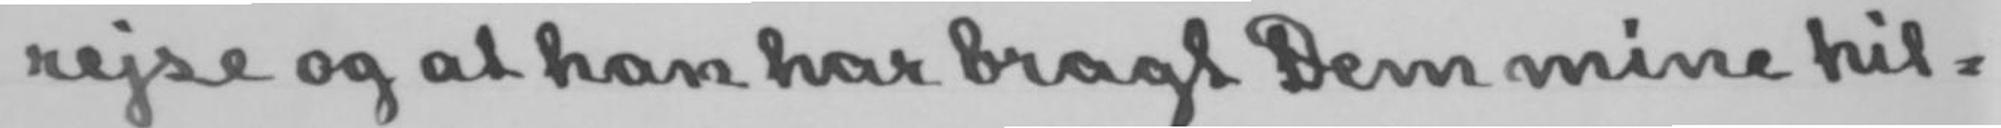rejse og at han har bragt Dem mine hil-
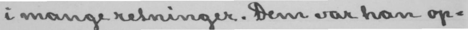i mange retninger. Dem var han op-
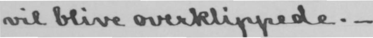vil blive overklippede.-
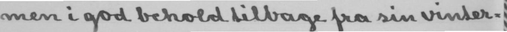men i god behold tilbage fra sin vinter-
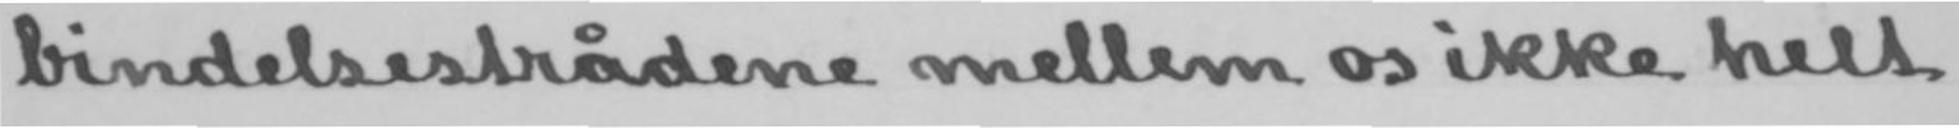bindelsestrådene mellem os ikke helt
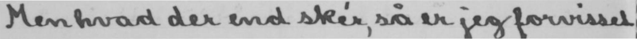Men hvad der end skér, så er jeg forvisset
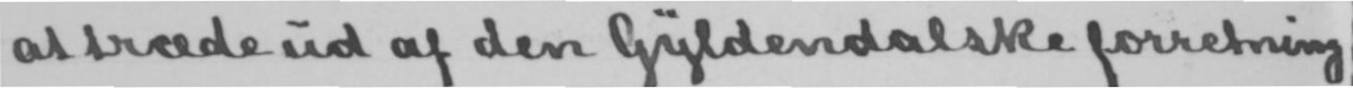at træde ud af den Gyldendalske forretning.
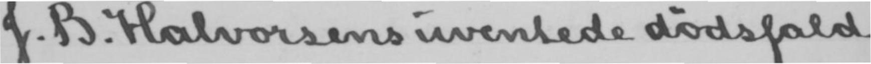J. B. Halvorsens uventede dødsfald
